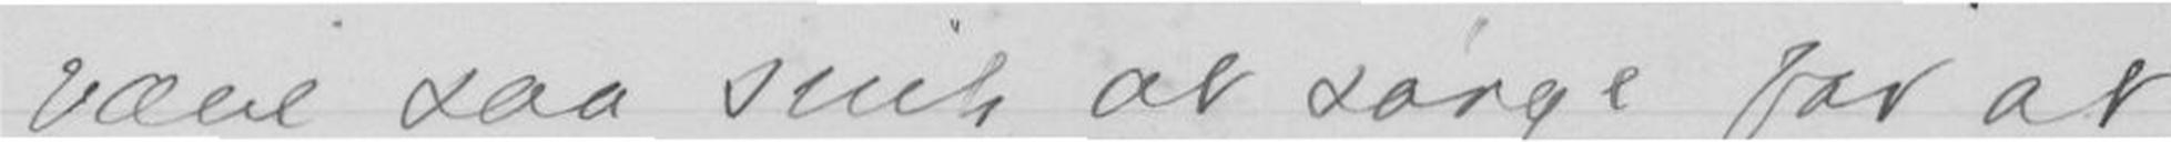være saa snil, at sørge for at
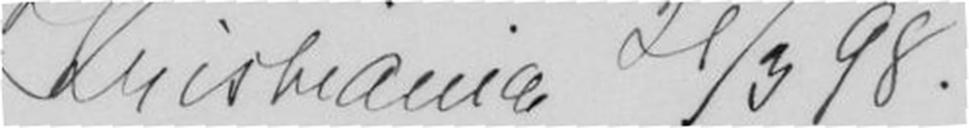Kristiania 21/3 98
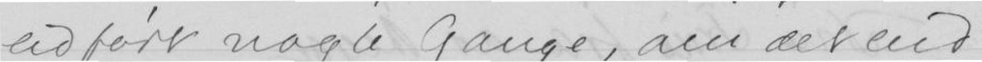udføre nogle Gange, om det end
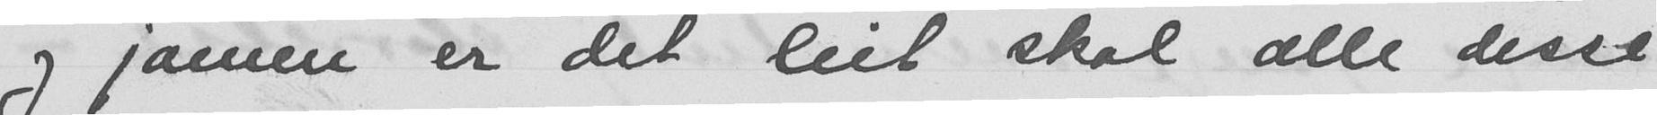og jamen er det leit skal alle disse
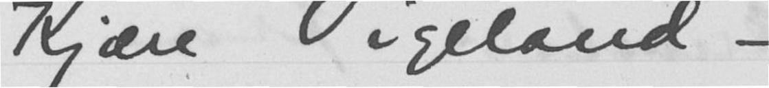Kjære Vigeland —
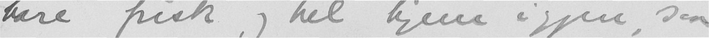bare frisk og hel hjem igjen, saa
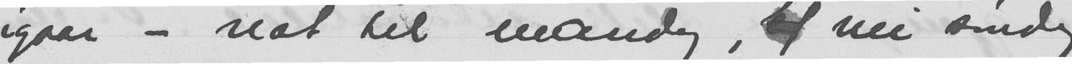igaar - nat til mandag,  nei søndag
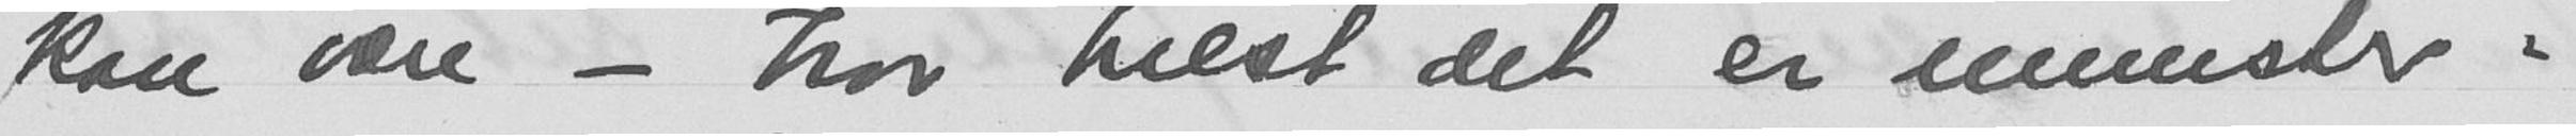kan vere - tror helst det er minister-
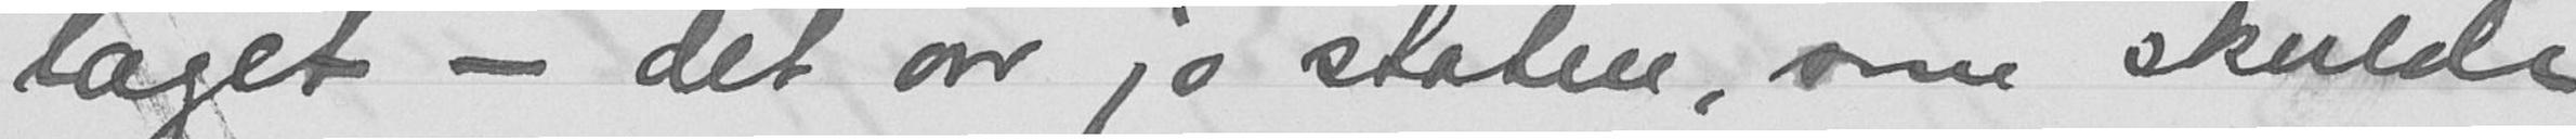laget - det er jo staten, som skulde
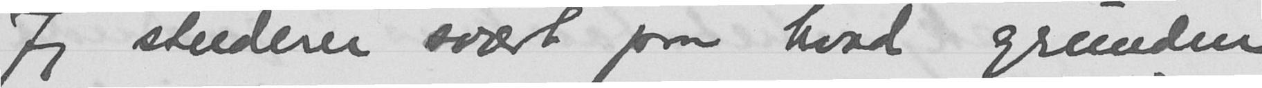Jeg studerer svært paa hvad grunden
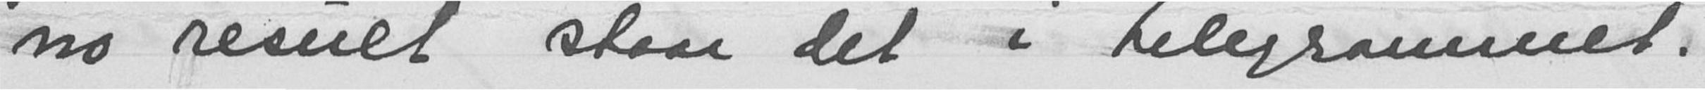no result staar det i telegrammet.
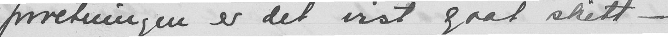forretningen er det vist gaat skitt -
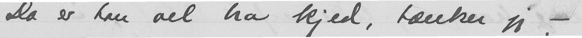Da er han vel bra kjed, tænker jeg -
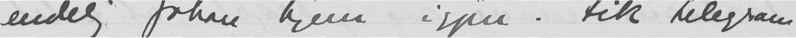endelig Johan hjem igjen. Fik telegram
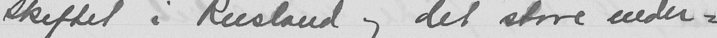skiftet i Rusland og det store neder-
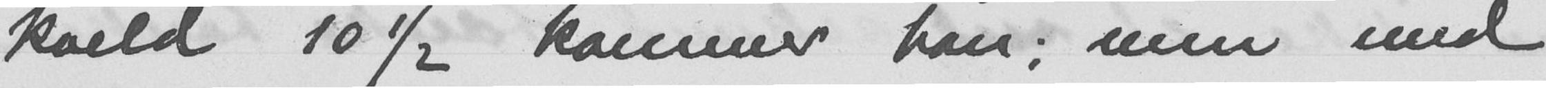kveld 10 1/2 kommer han; men med
